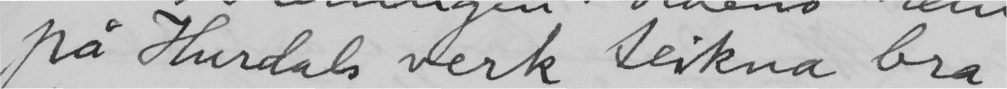på Hurdals verk teikna bra
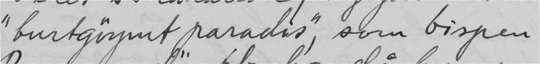"bortgöymt paradis", som bispen
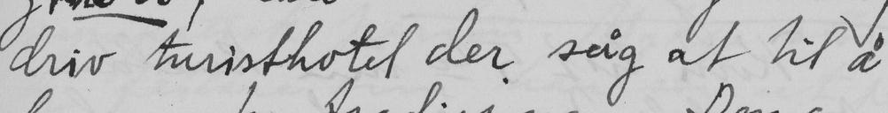driv turisthotel der såg at til å
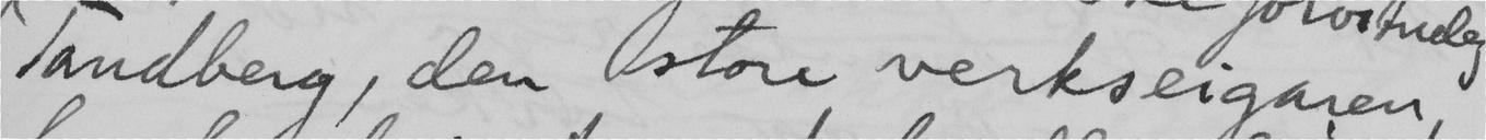Tandberg, den store verkseigaren,
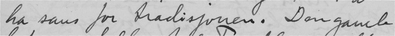ha sans for tradisjonen. Den gamle
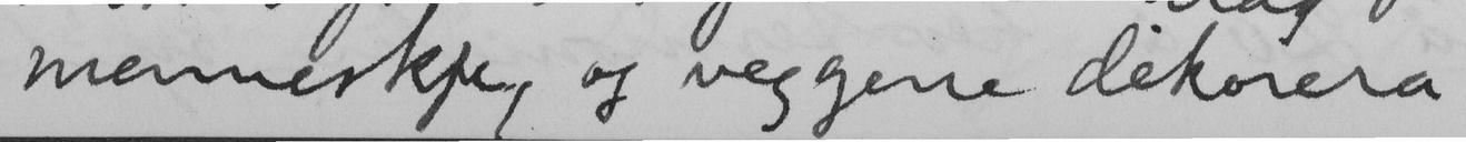menneskje, og veggene dekorera
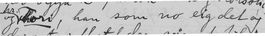og hr. Thori, han som no eig det og
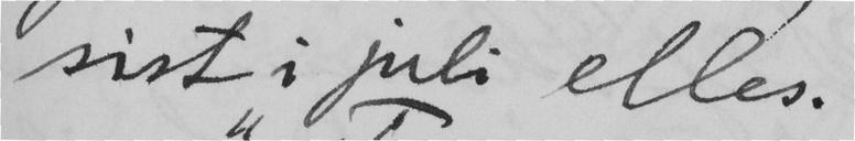sist i juli elles.
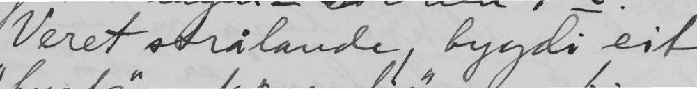Været strålande, bygdi eit
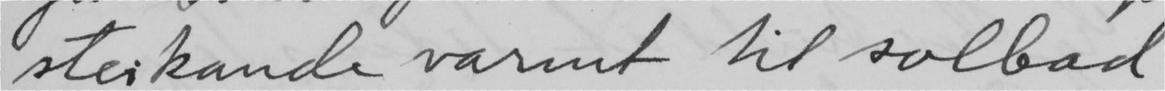steikande varmt til solbad
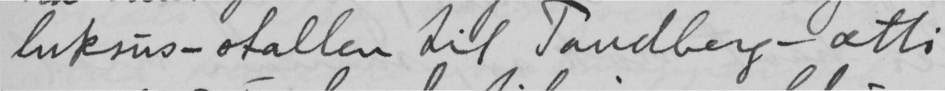luksus-stallen til Tandberg - atti
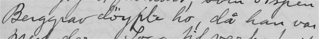Berggrav döypte ho då han var
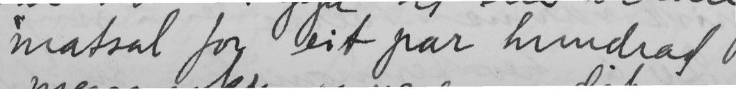matsal for eit par hundrad
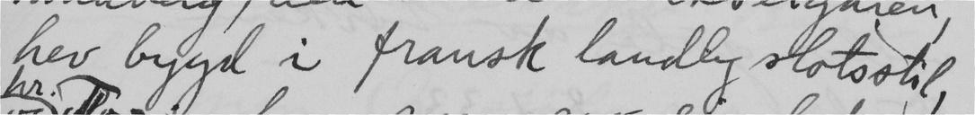hev bygd i fransk landlig slotsstil,
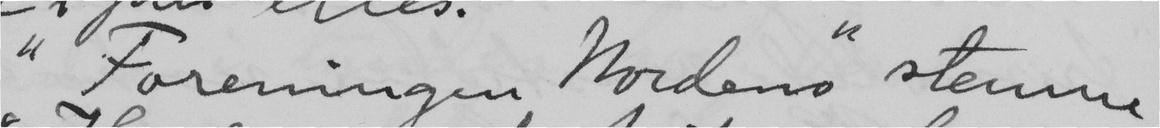"Foreningen Nordens" stemne
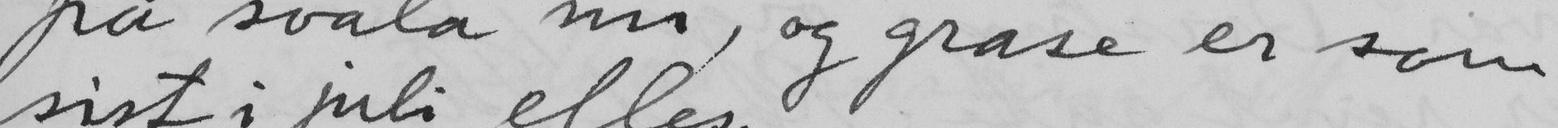på svala mi, og grase er som
